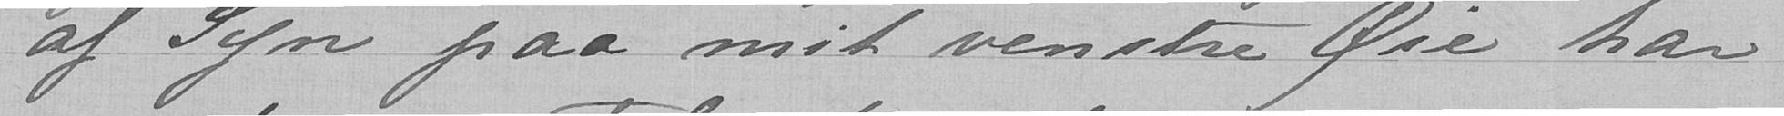af Syn paa mit venstre Øie har
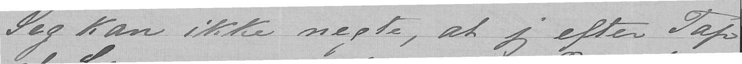Jeg kan ikke negte, at jeg efter Tap
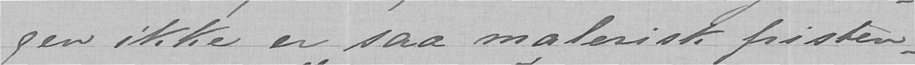gen ikke er saa malerisk fristen-
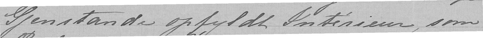Gjenstande opfyldt Intérieur, som
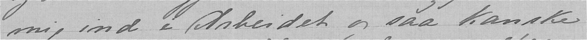mig ind i Arbeidet, og saa kanske
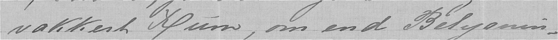vakkert Rum, om end Belysnin-
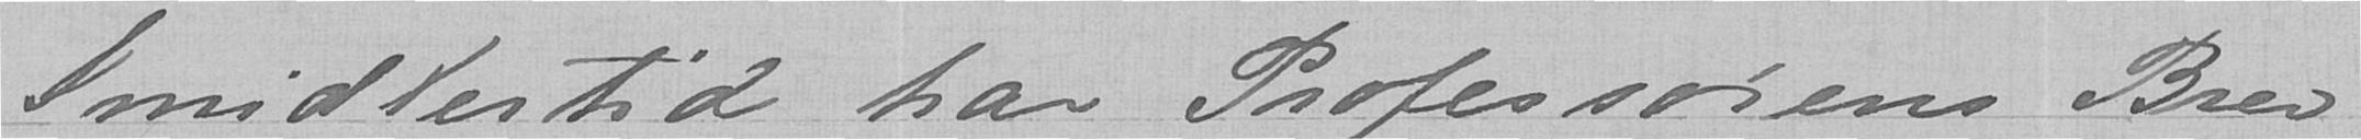Imidlertid har Professorens Brev
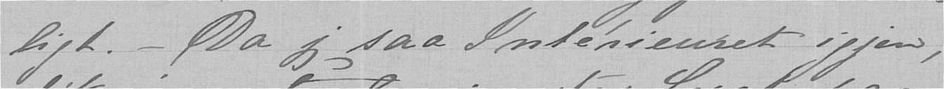ligt. - Da jeg saa Intérieuret igjen,
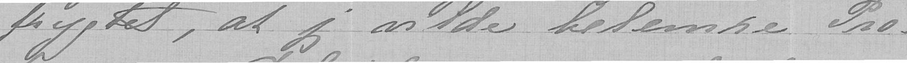frygtet, at jeg vilde belemre Pro-
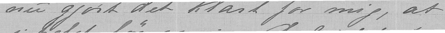nu gjort det klart for mig, at
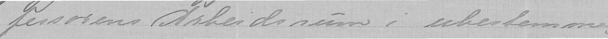fessorens Arbeidsrum og ubestemme-
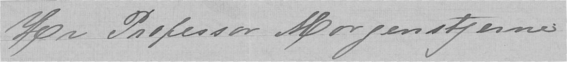Hr Professor Morgenstjerne
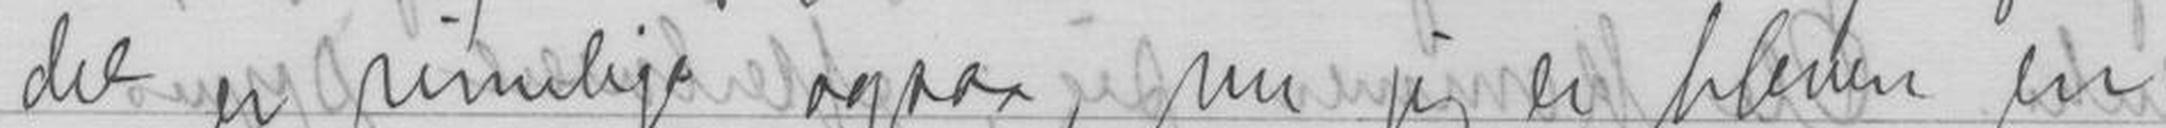det er rimeligt ogsaa, nu jeg er bleven en
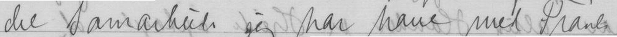det Samarbeide jeg har havt med Frants
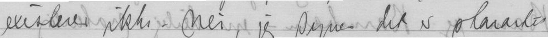existerer ikke. Nei, jeg synes det er storartet
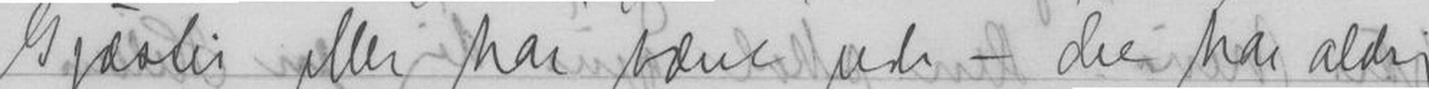Gjæster eller har været ude - det har aldrig
